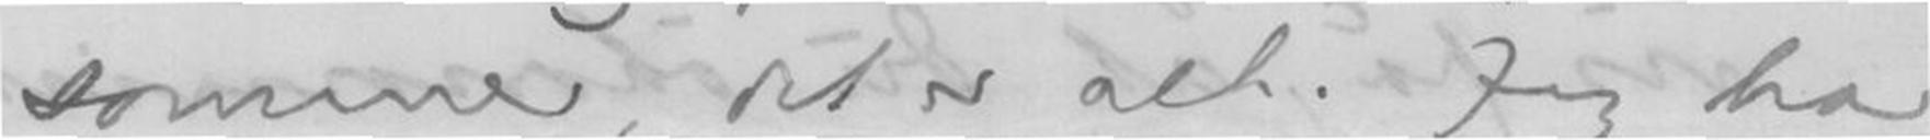sommer, det er alt. Jeg har
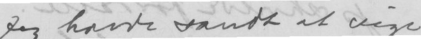Jeg havde sandt at sige
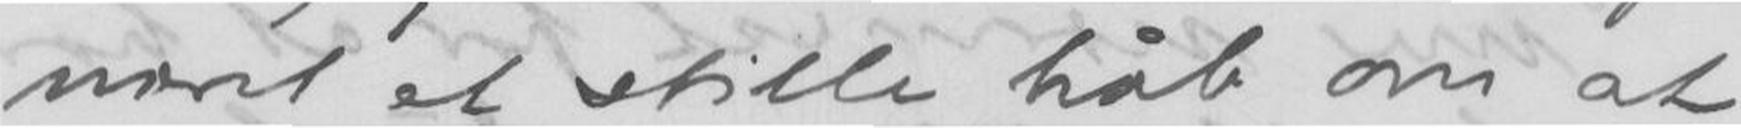næret et stille håb om at
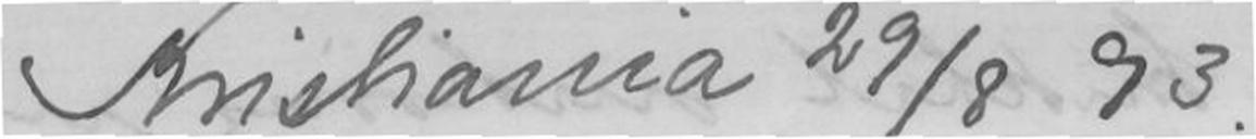Kristiania 29/8 93
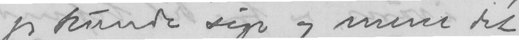jeg kunde sige og mene det
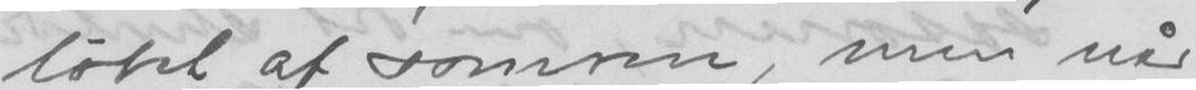løbet af sommeren,men når
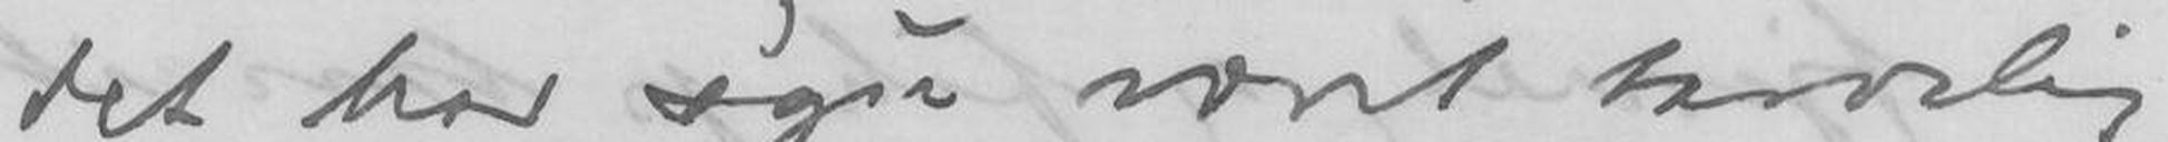det har s'gu været tarvelig.
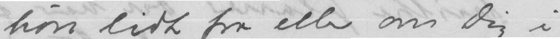høre lidt fra eller om dig i
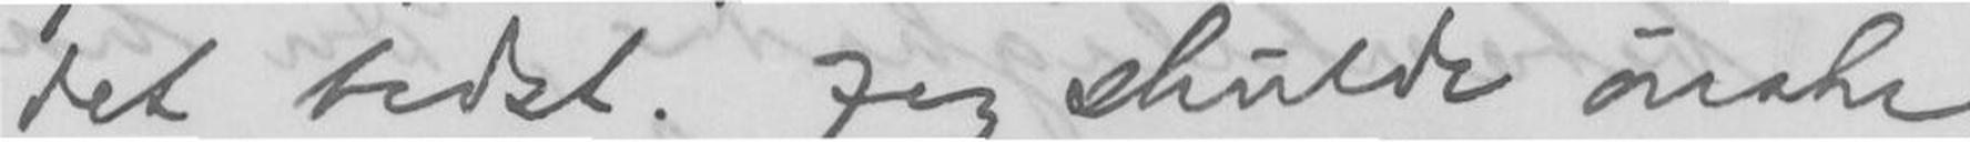det bedst. Jeg skulde ønske,
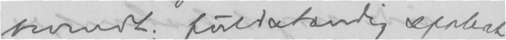besvendt; fuldstændig spoleret
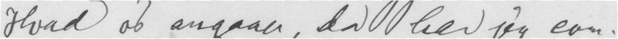Hvad os angaaer, da har jeg com-
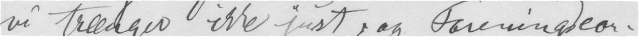vi trænger ikke just, og Foreningscon-
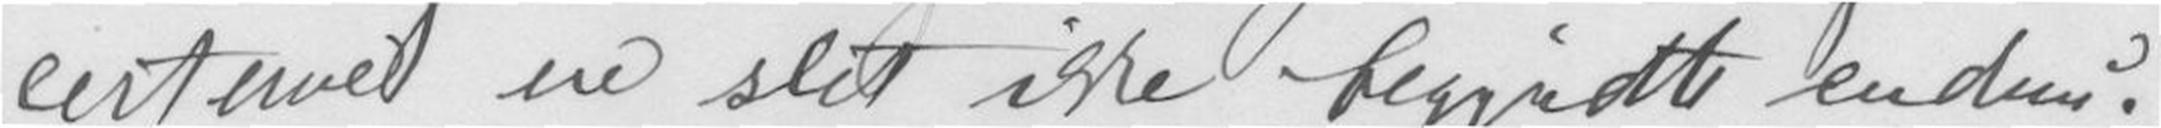certerne ere slet ikke begyndte endnu?
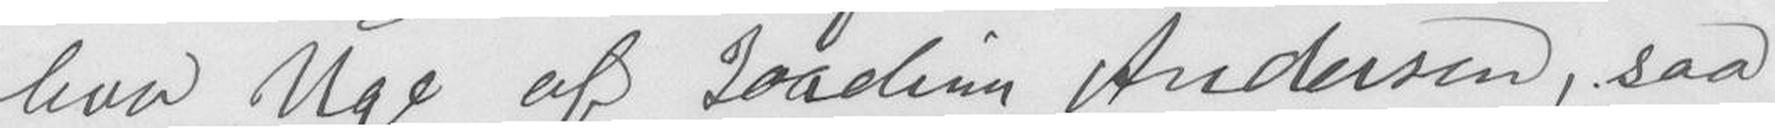hver Uge af Joachim Andersen, saa
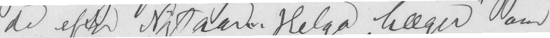de efter Nytaar. Helga Læger om
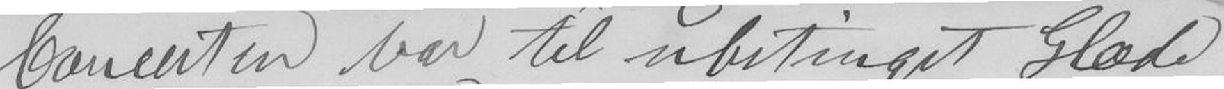Concerten var til ubetinget Glæde
